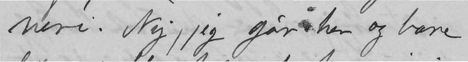neri. Nej, jeg går her og bare
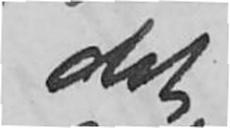det
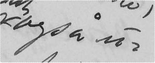også u-
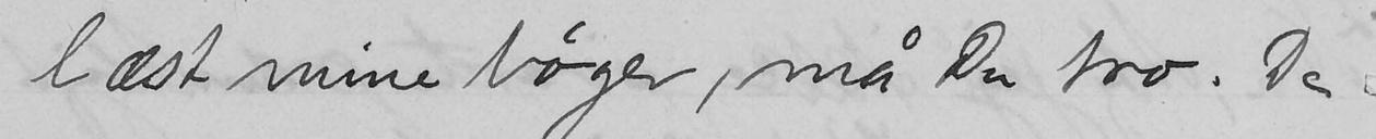læst mine bøger, må Du tro. De
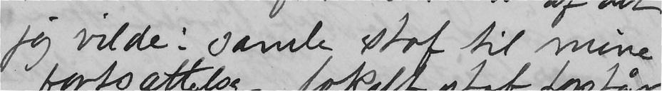jeg vilde: samle stof til mine
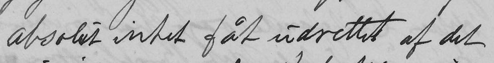absolut intet fåt udrettet af det
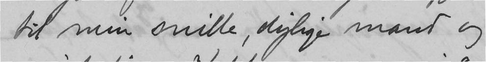til min snille, dejlige mand og
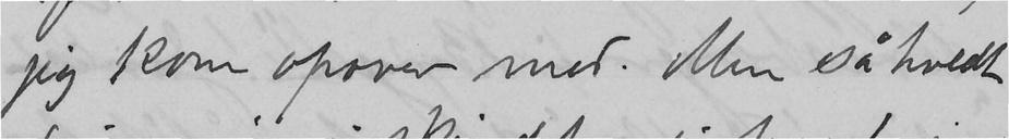jeg kom opover med. Men så holdt
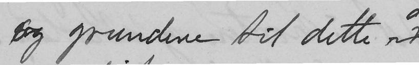og grundene til dette er
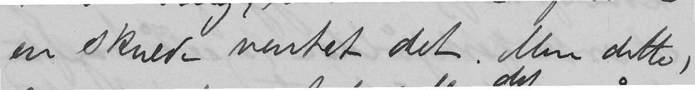en skulde ventet det. Men dette,
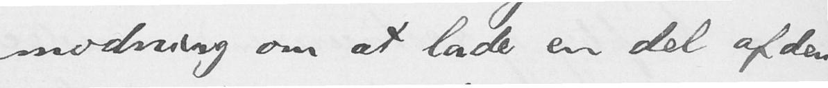modning om at lade en del af den
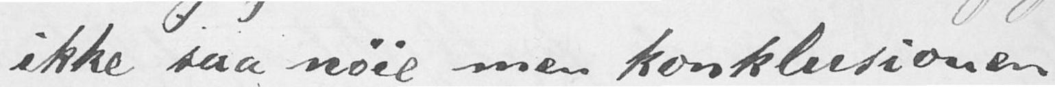ikke saa nøie, men konklusionen
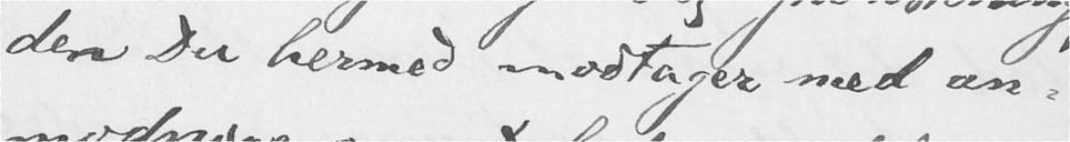den Du hermed modtager med an-
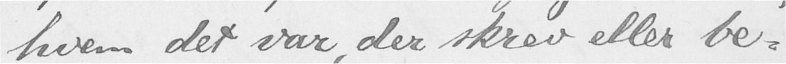hvem det var, der skrev eller be-
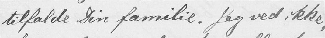tilfalde Din familie. Jeg ved ikke,
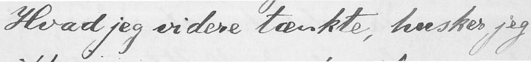Hvad jeg videre tænkte, husker jeg
